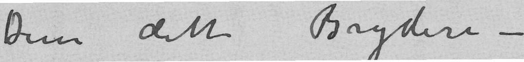Dem dette Bryderi –
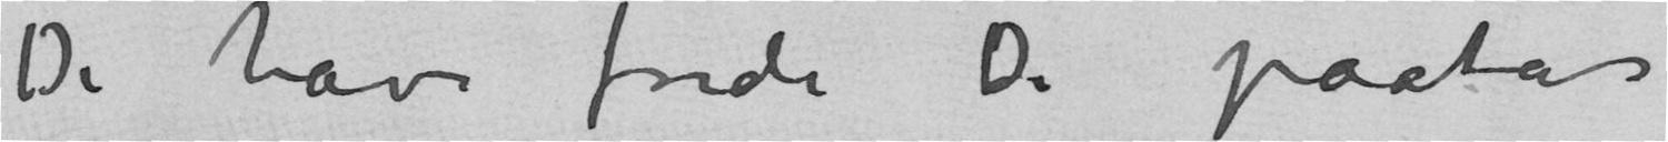De have fordi De paatar
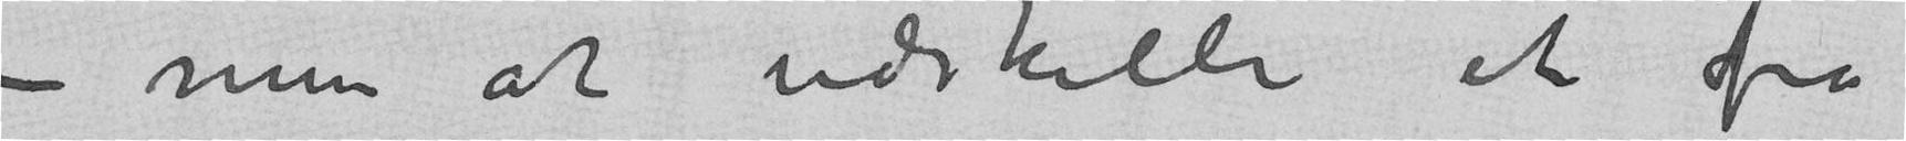– men at udskille et ifra
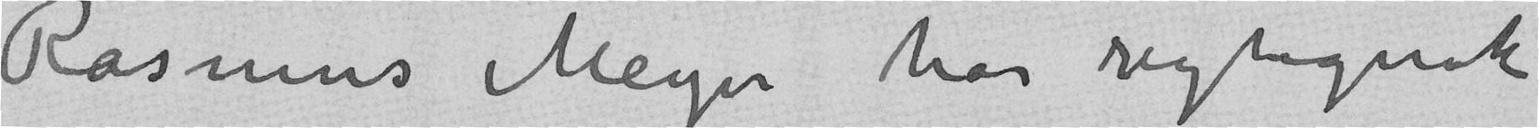Rasmus Meyer har rigtignok
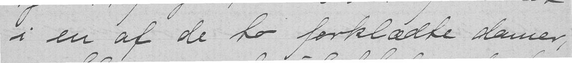i en af de to forklædte damer,
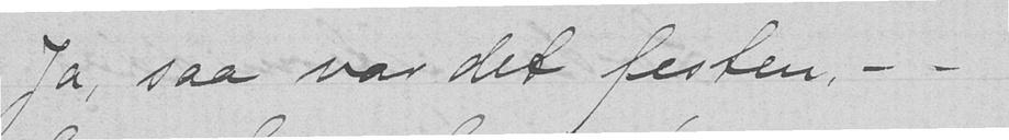Ja, saa var det festen. - -
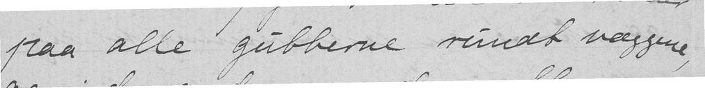paa alle gubberne rundt væggene,
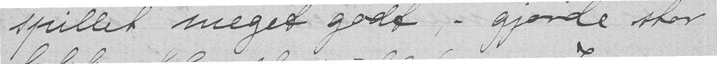spillet meget godt, - gjorde stor
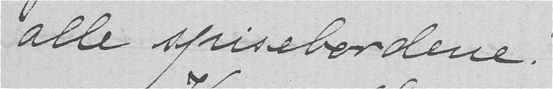alle spisebordene.
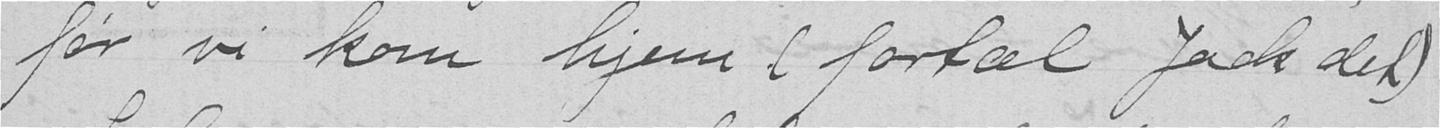før vi kom hjem (fortal Jack det)
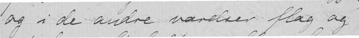og i de andre værelser flag og
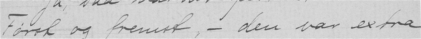Først og fremst, - den var extra
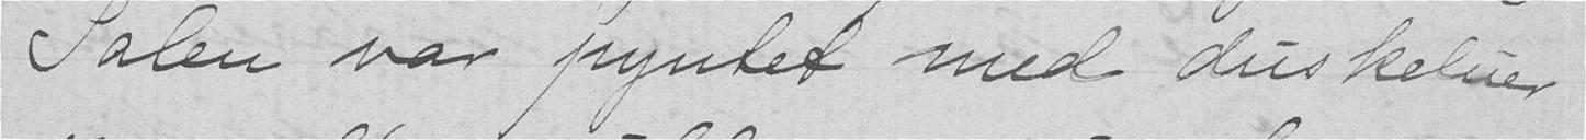Salen var pyntet med duskeluer
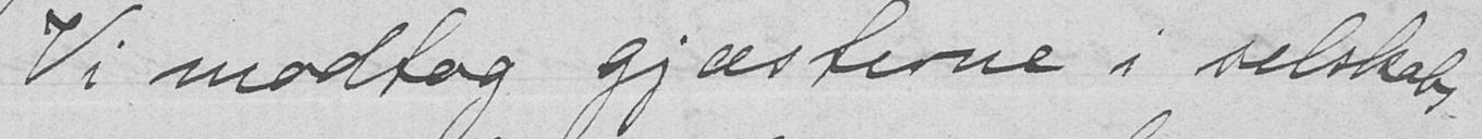Vi modtog gjæsterne i selskabs-
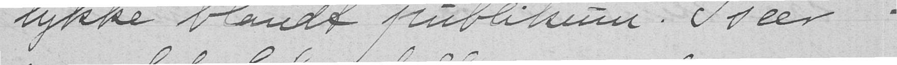lykke blandt publikum. Især
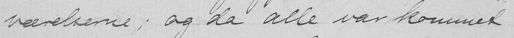værelserne; og da alle var kommet
